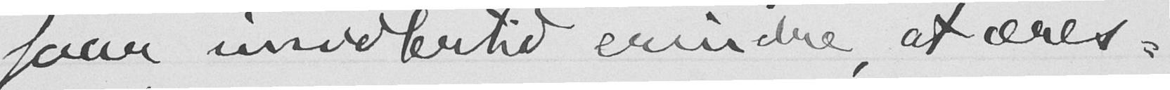faar imidlertid erindre, at æres-
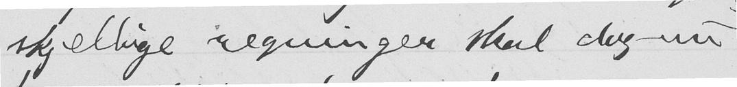skjellige regninger skal dog nu
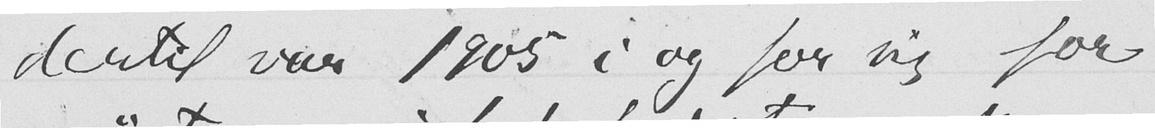dertil var 1905 i og for sig for
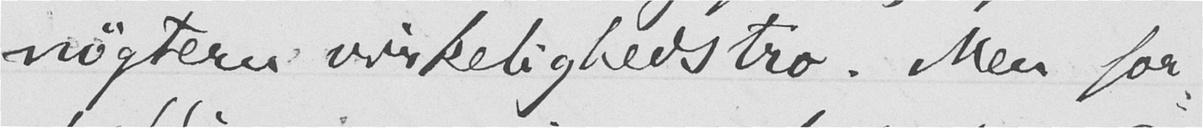nøgtern virkelighedstro. Men for-
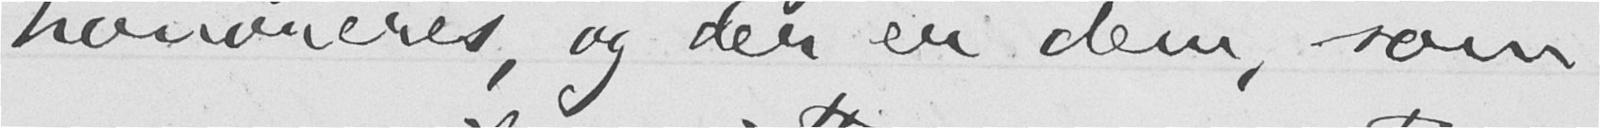honoreres, og der er dem, som
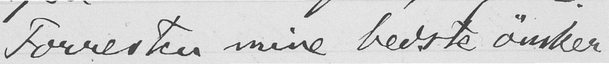Forresten mine bedste ønsker
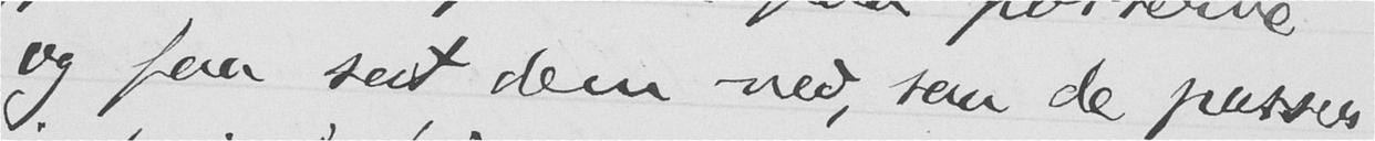og faa sat dem ned, saa de passer
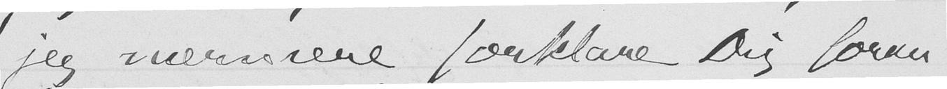jeg nærmere forklare Dig foran-
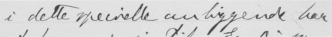i dette specielle anliggende har
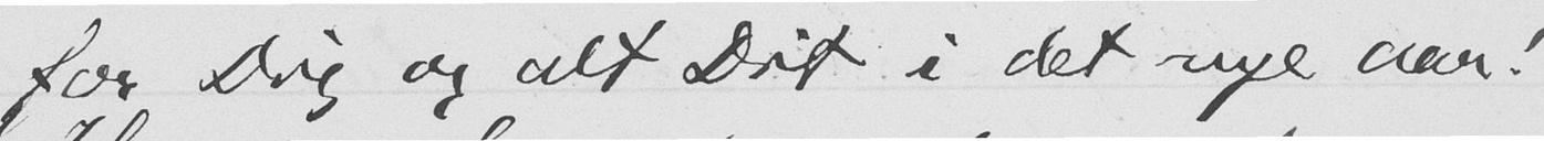for Dig og alt Dit i det nye aar!
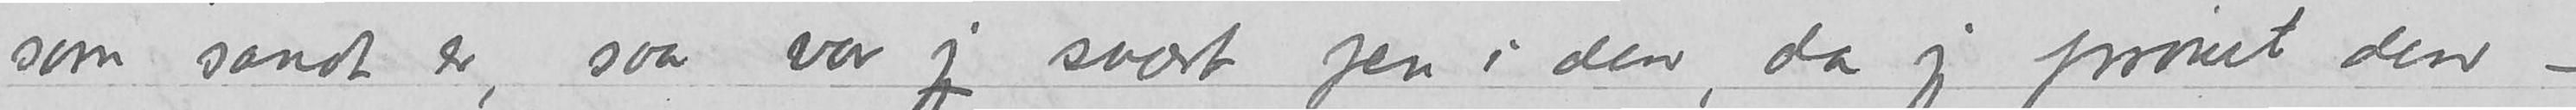som sandt er, saa var jeg svært pen i den, da jeg prøvet den –
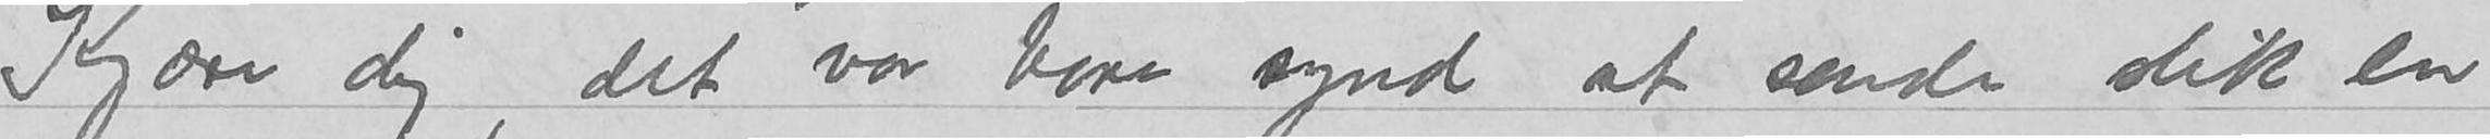Kjære dig, det var bare synd at sende slik en
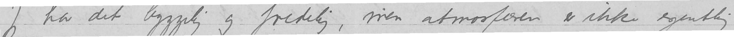Jeg har det hyggelig og fredelig, men atmosfæren er ikke egentlig
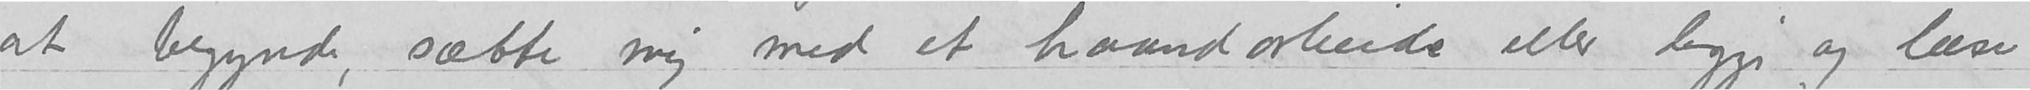at begynde, sætte mig med et haandarbeide eller ligge og læse
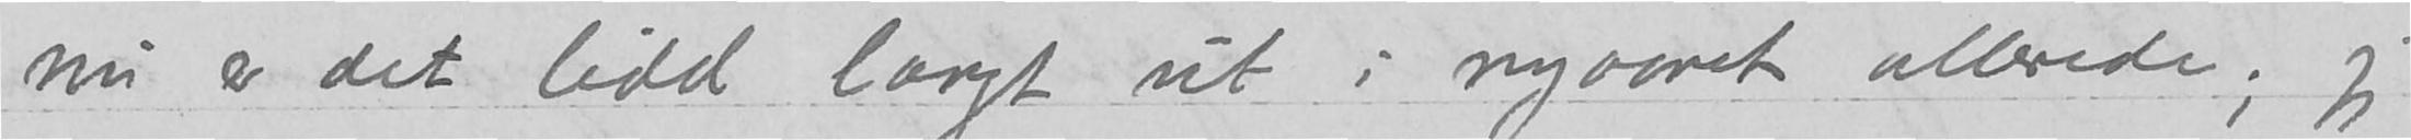Nu er det lidd langt ut i nyaaret allerede; jeg
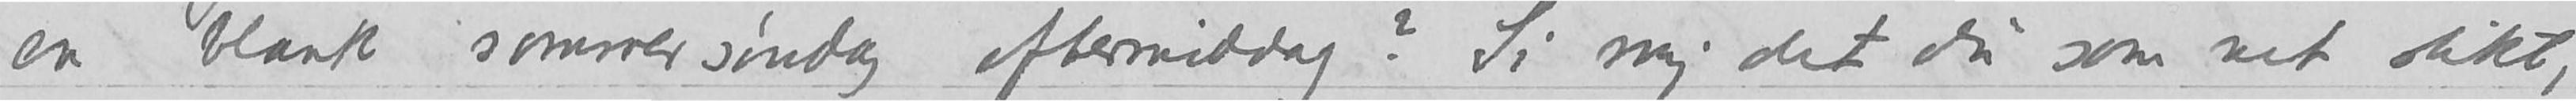en blank sommersøndag eftermiddag?  Si mig det du som vet slikt
